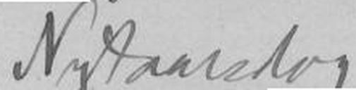Nytaarsdag
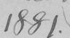1881.
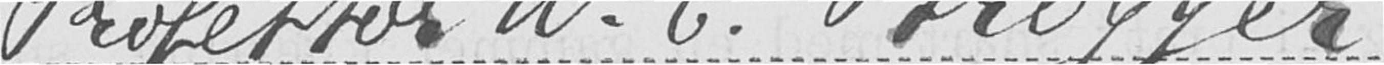Professor W. C. Brøgger
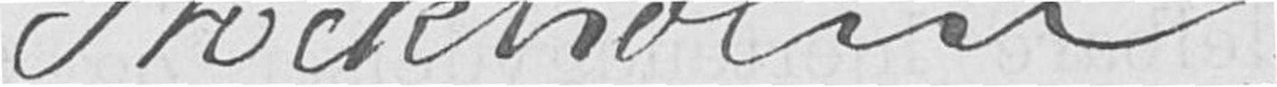Stockholm.
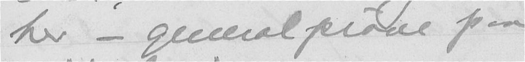her - generalprøve paa
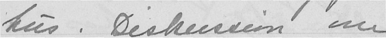hus. Diskussion om
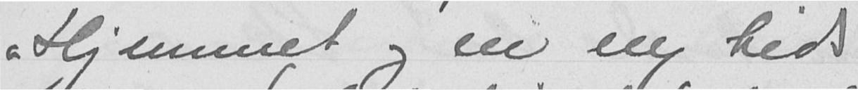"Hjemmet og en ny tids
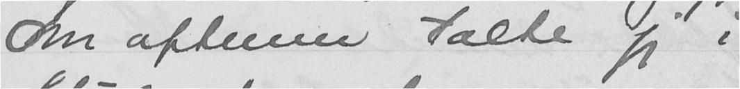Om aftenen talte jeg i
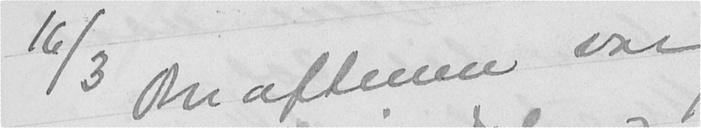16/3 Om aftenen var
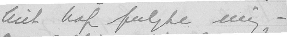Mit hof fulgte mig -
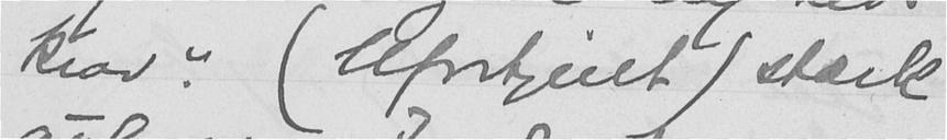krav". (Ufortjent) stærk
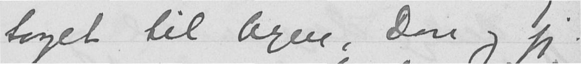toget til byen, Don og jeg.
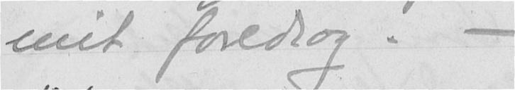mit foredrag. -
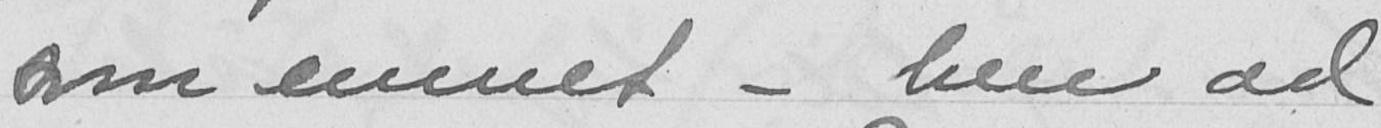som emnet - hen ad
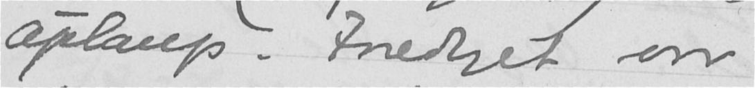aplaup. Foredraget var
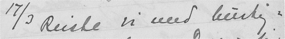17/3 Reiste vi med hurtig-
